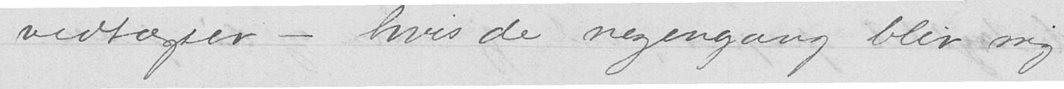vedtægter - hvis de nogengang blir mig
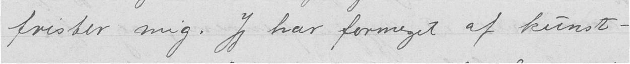frister mig. Jeg har formeget af kunst-
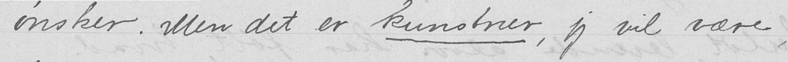ønsker. Men det er kunstner, jeg vil være,
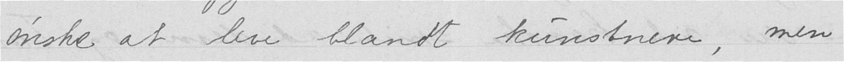ønske at leve blandt kunstnere, men
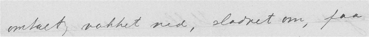omtalt, rakket ned, sladret om, faa
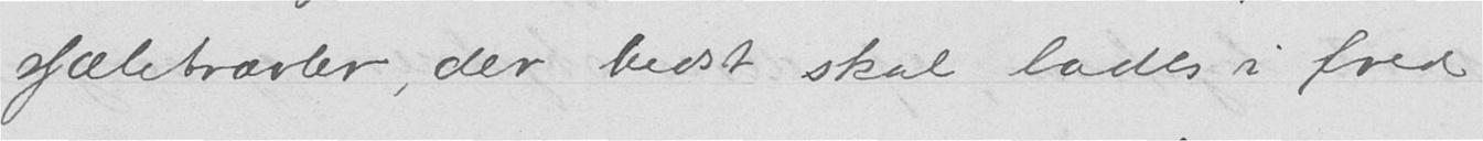sjæletrævler, der bedst kan lades i fred,
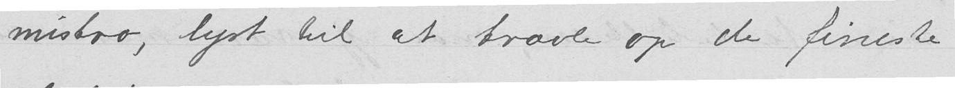mistro, lyst til at trævle op de fineste
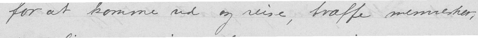for at komme ud og reise, træffe mennesker,
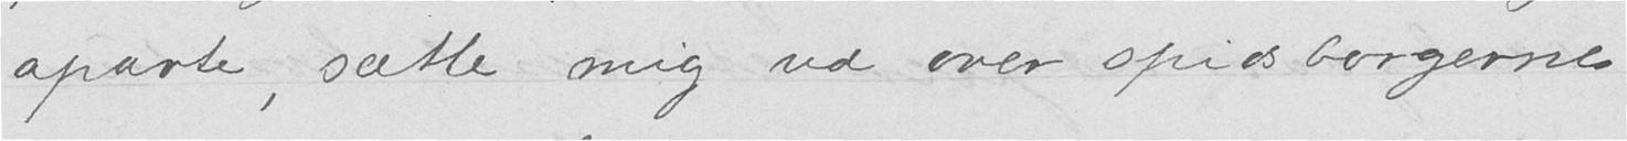aparte, sætte mig ud over spidsborgernes
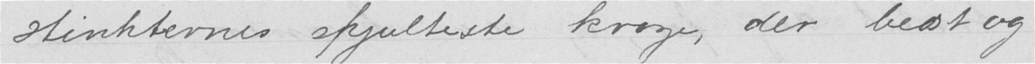stinkternes skjulteste kroger, der bedst og
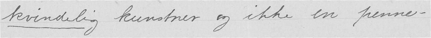kvindelig kunstner og ikke en penne-
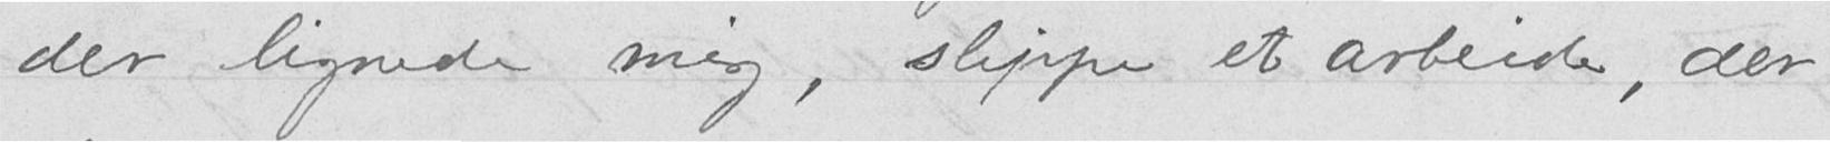der lignede mig, slippe et arbeide, der
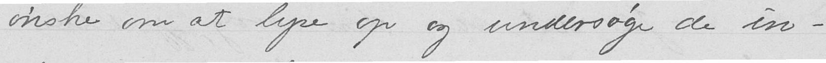ønske om at lyse op og undersøge de in-
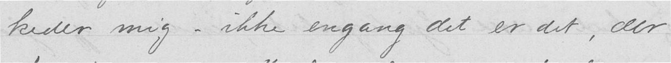keder mig - ikke engang det er det, der
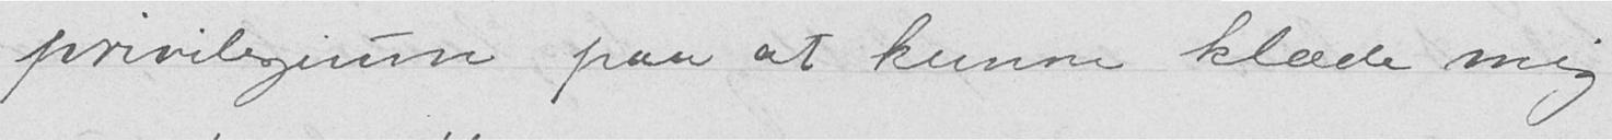privilegium paa at kunne klæde mig
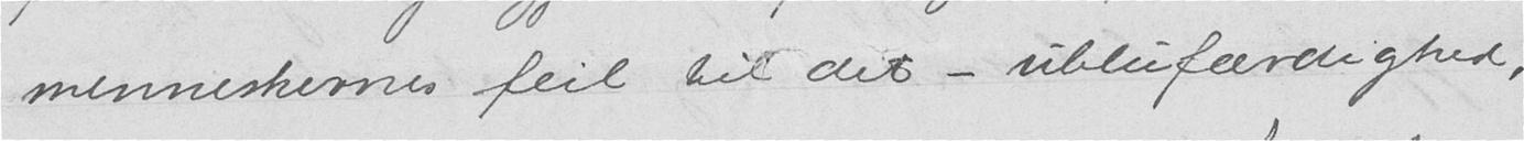menneskernes feil til det - ublufærdighed,
